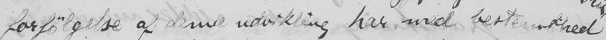forfølgelse af denne udvikling har med bestemthed
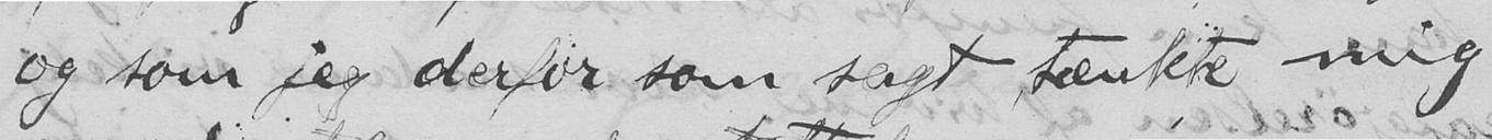og som jeg derfor som sagt tænkte mig
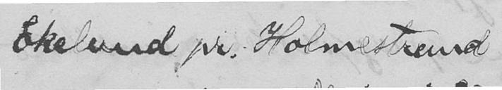Ekelund pr. Holmestrand
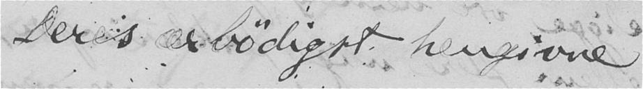Deres ærbødigst hengivne
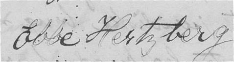Ebbe Hertzberg.
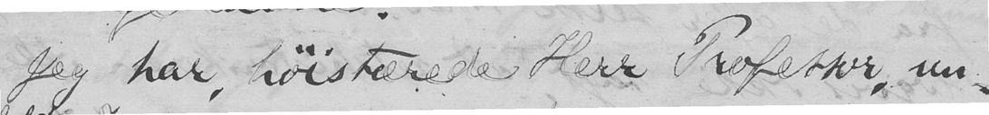Jeg har, høistærede Herr Professor, un-
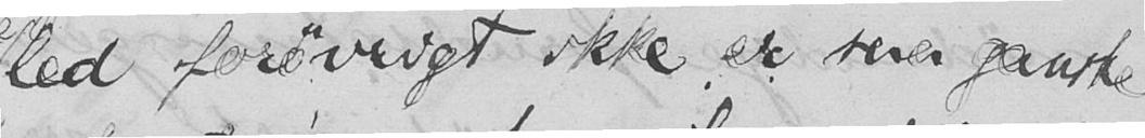led forøvrigt ikke er saa ganske
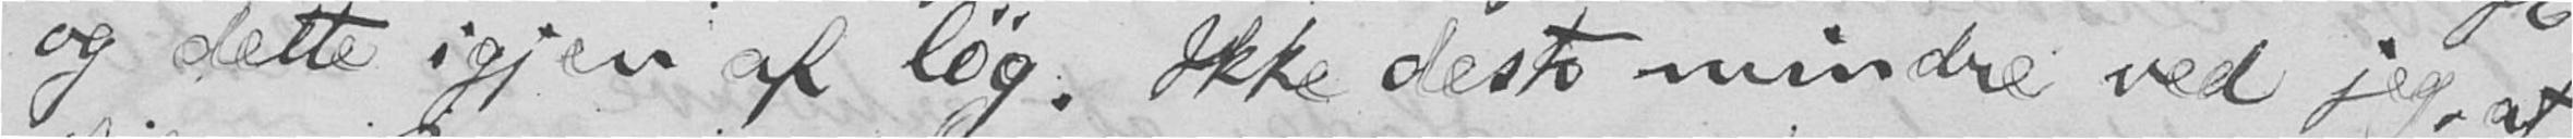og dette igjen af løg. Ikke desto mindre ved jeg, at
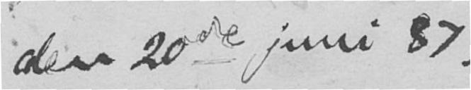den 20de juni 87.
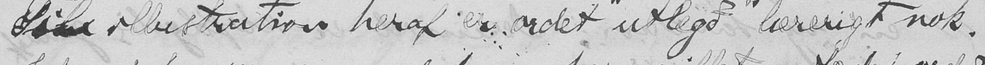Til illustration heraf er ordet "utlegd" lærerigt nok.
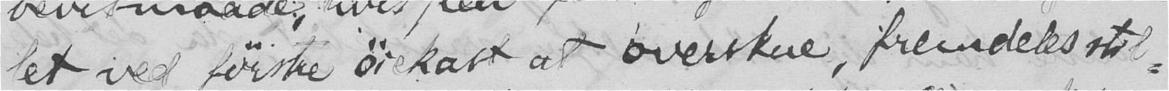let ved førstre øiekast at overskue, fremdeles stil-
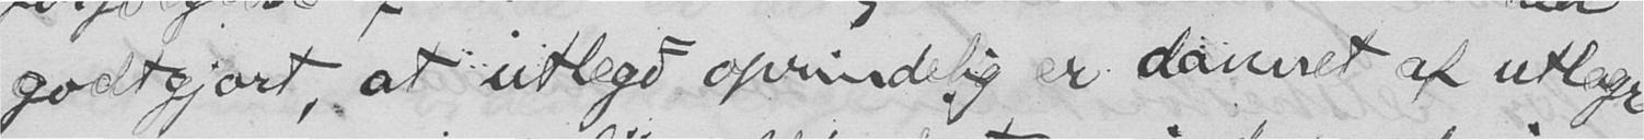godtgjort, at utlegd oprindelig er dannet af utlagr
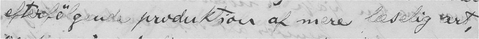efterfølgende produktion af mere læselig art,
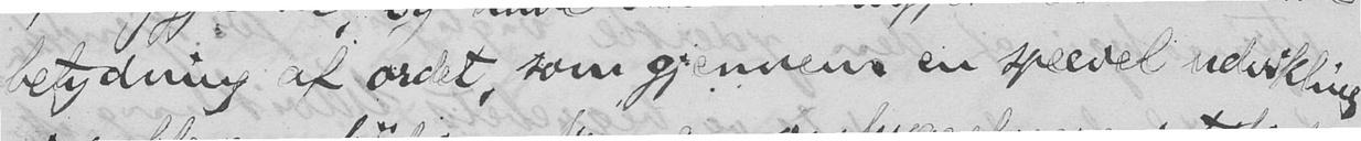betydning af ordet, som gjennem en speciel udvikling
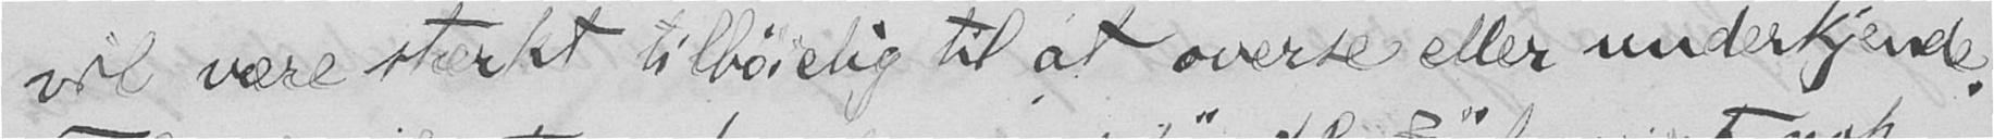vil være stærkt tilbøielig til at overse eller underkjende.
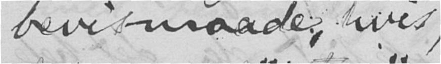bevismaade, hvis
